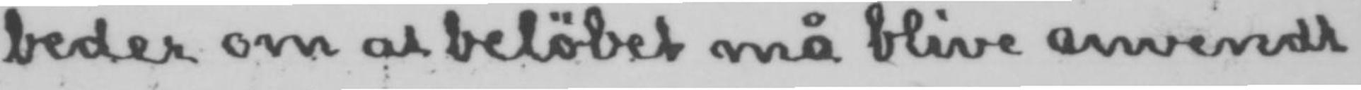beder om at beløbet må blive anvendt
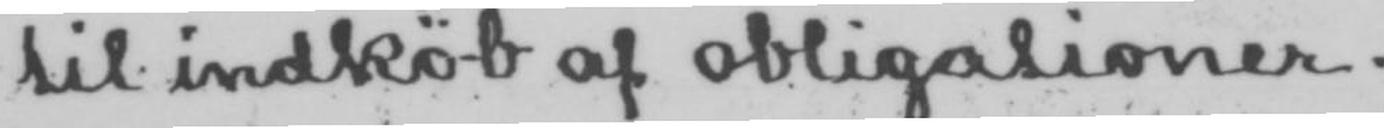til indkøb af obligationer.
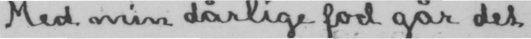Med min dårlige fod går det
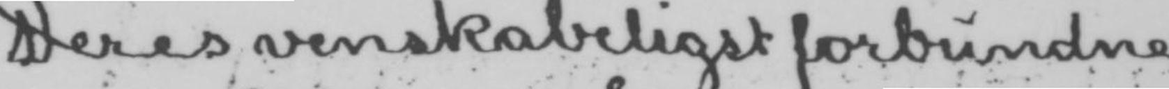Deres venskabeligst forbundne
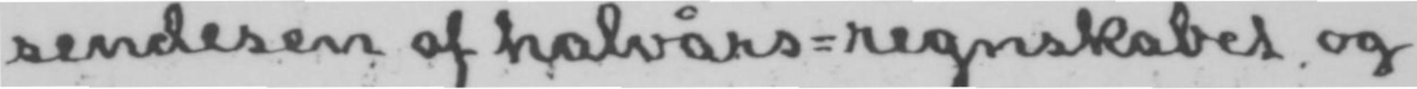sendesen af halvårs-regnskabet og
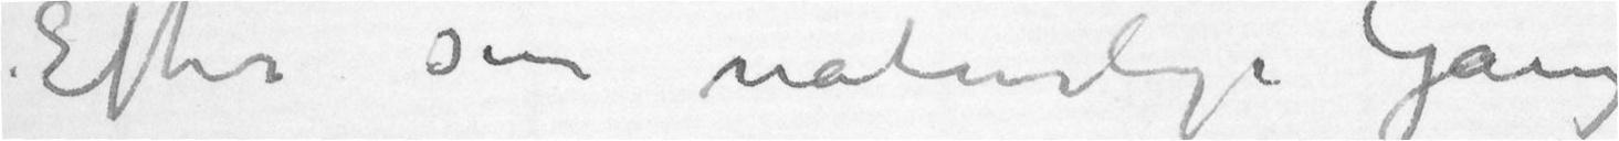Efter sin naturlige Gang
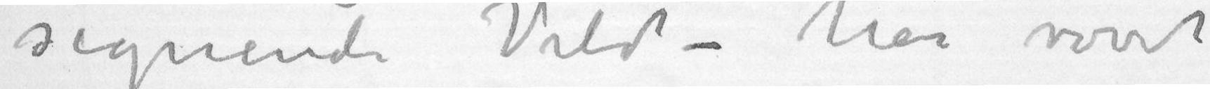segnende Vildt – har vovet
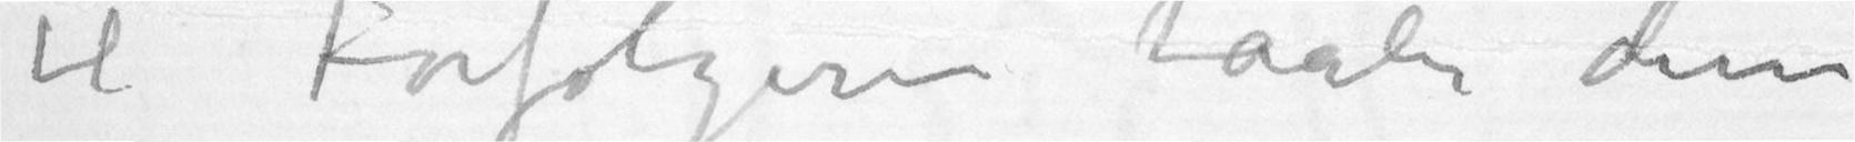til Forfølgerne taaler disse
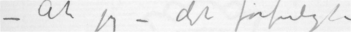–	At jeg – det forfulgte
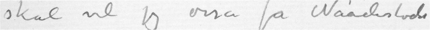skal vel jeg osså få Naadestødet
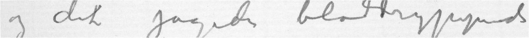og det jagede bloddryppende
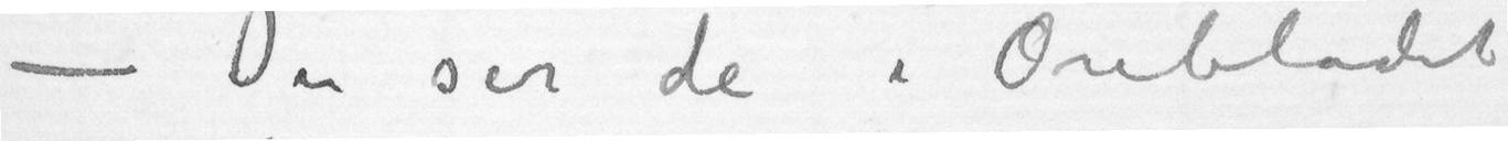– Du ser de i Ørebladet
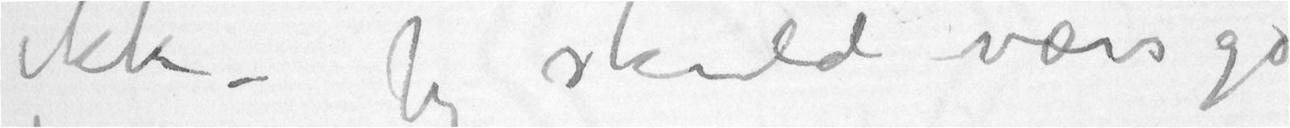ikke – Jeg skulde værsgo
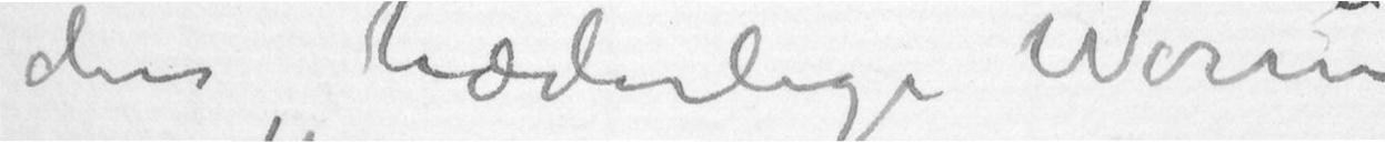dens «hæderlige Worm»
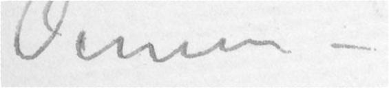Øinene –
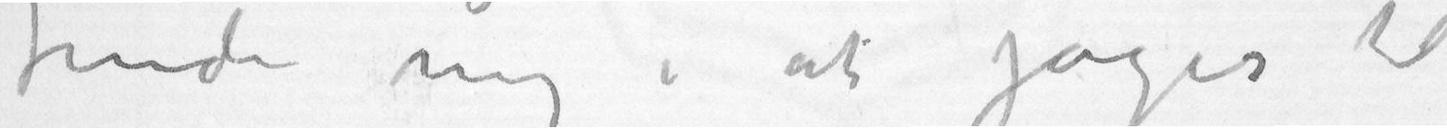finde mig i at jages til
